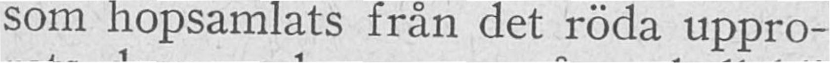som hopsamlats från det röda uppro-
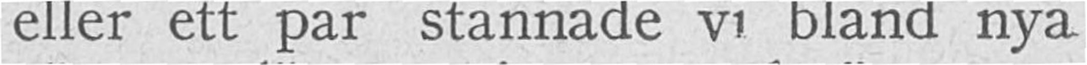eller ett par stannade vi bland nya
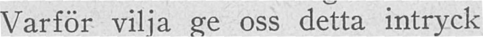Varför vilja ge oss detta intryck
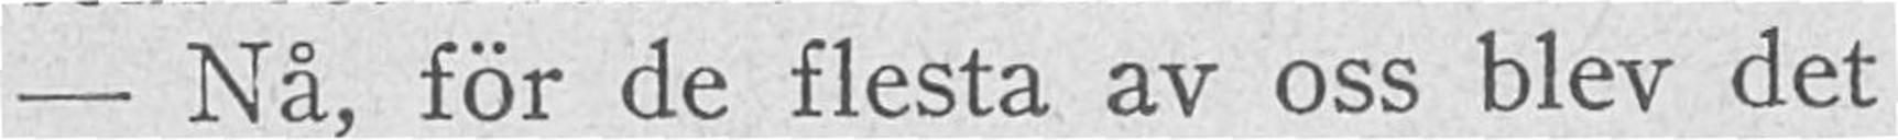- Nå, för de flesta av oss blev det
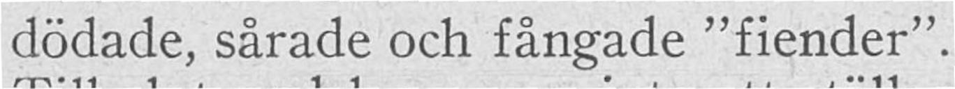dödade, sårade och fångade "fiender".
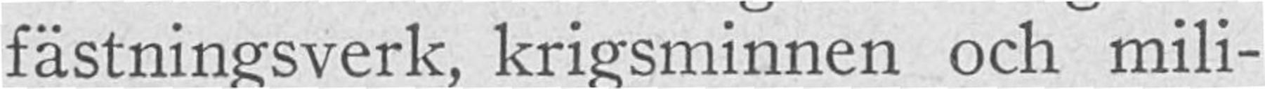fästningsverk, krigsminnen och mili-
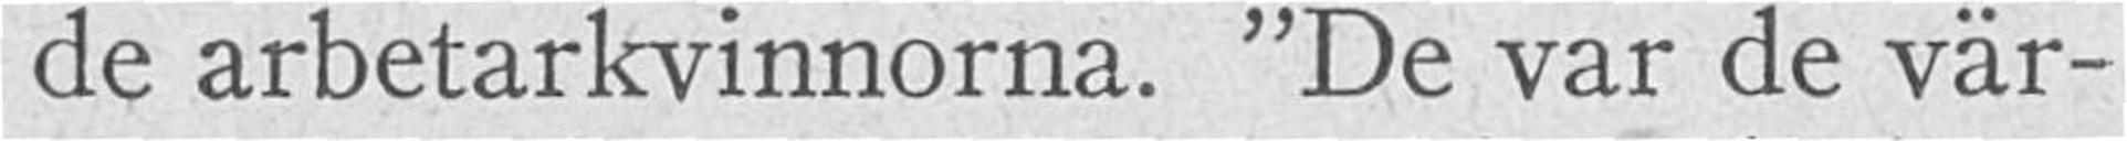de arbetarkvinnorna. "De var de vär-
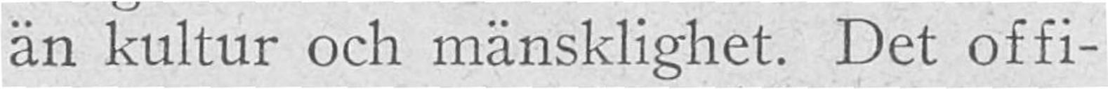än kultur och mänsklighet. Det offi-
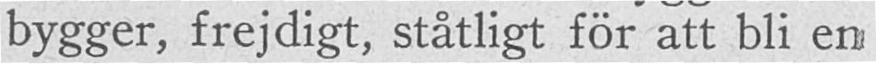bygger, frejdigt, ståtligt för att bli en
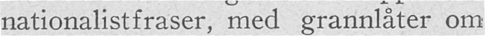nationalistfraser, med grannlåter om
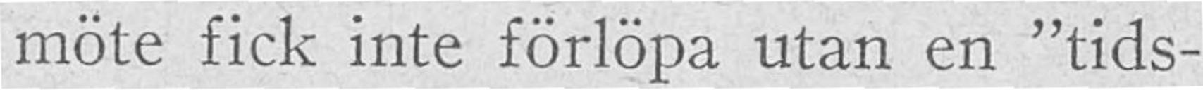möte fick inte förlöpa utan en "tids-
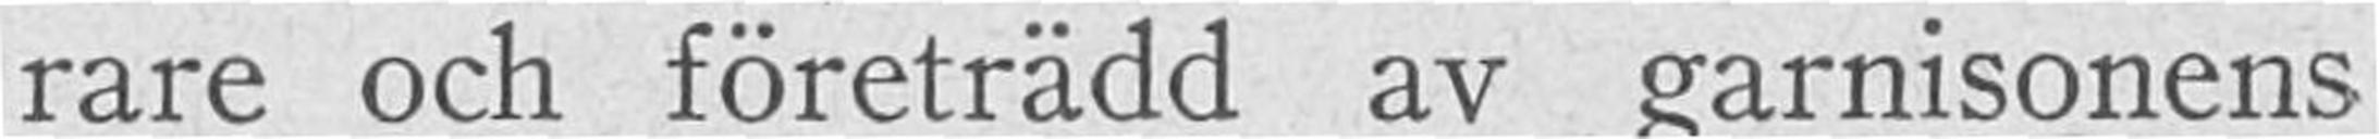rare och företrädd av garnisonens
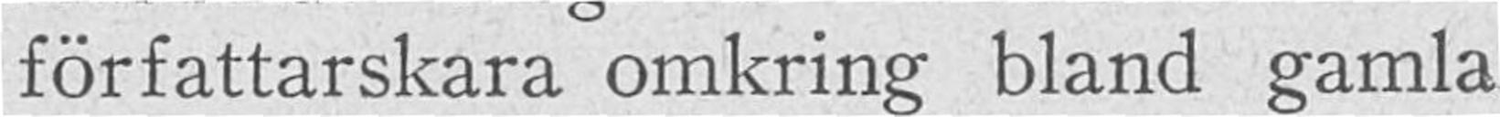författarskara omkring bland gamla
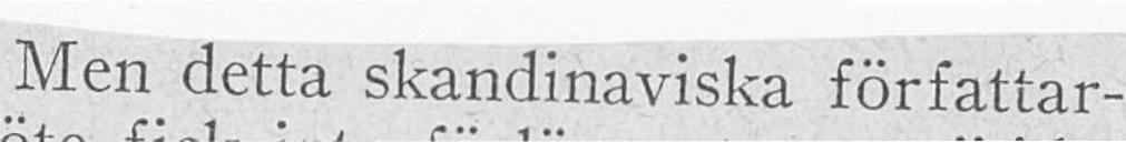Men detta skandinaviska författar-
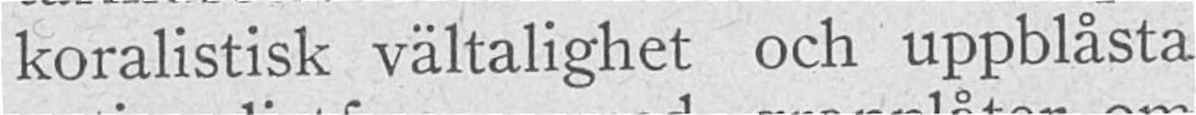koralistisk vältalighet och uppblästa
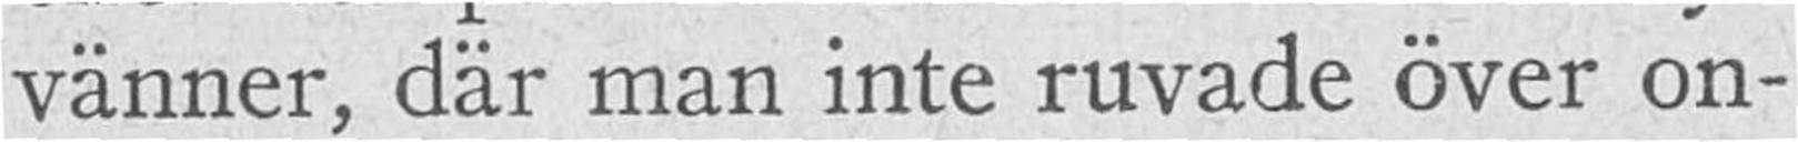vänner, där man inte ruvade över on-
